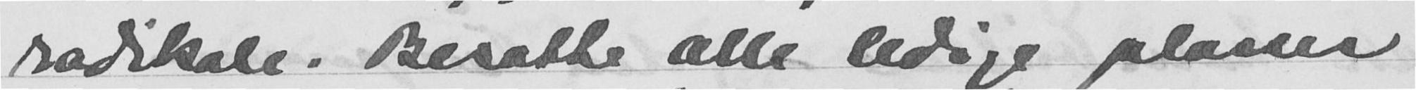radikale. Besatte alle ledige plasser
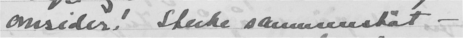omsider! Sterke sammenstøt -
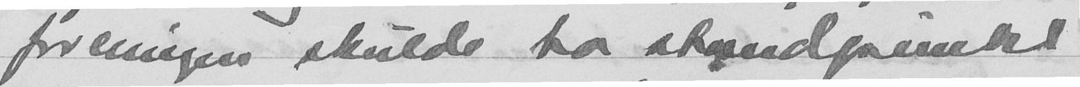foreningen skulde ta standpunkt
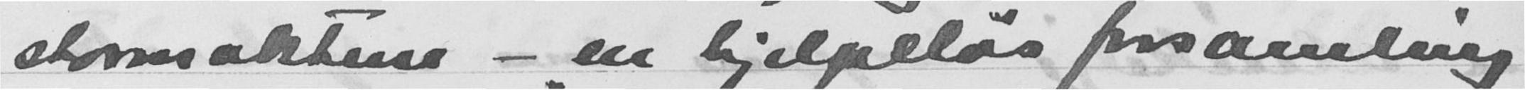stormaktene - en hjelpeløs forsamling
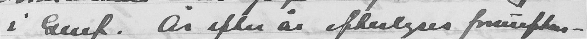i Genf. År efter år efterlyses fornuften -
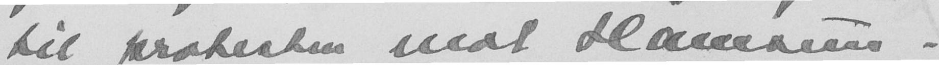til protesten mot Hamsun.
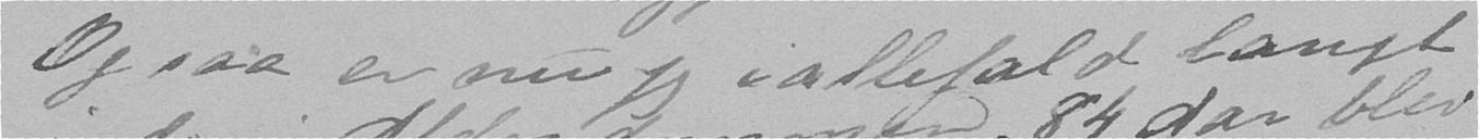Og saa er nu jeg iallefald langt
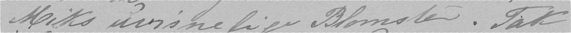Miks uvisnelige Blomster. Tak
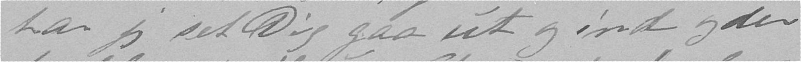har jeg set Dig gaa ut og ind og der
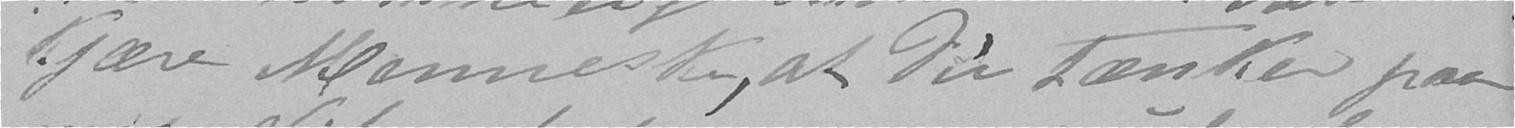kjære Menneske, at Du tænker paa
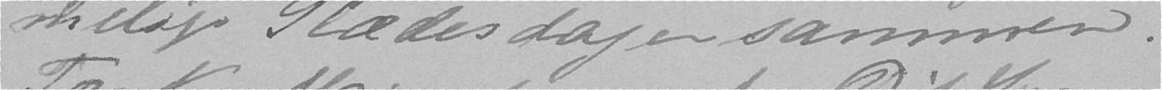melige Glædesdager sammen.
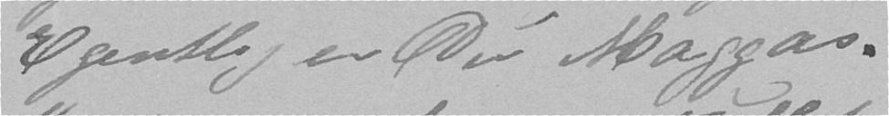Egentlig er Du Maggas.
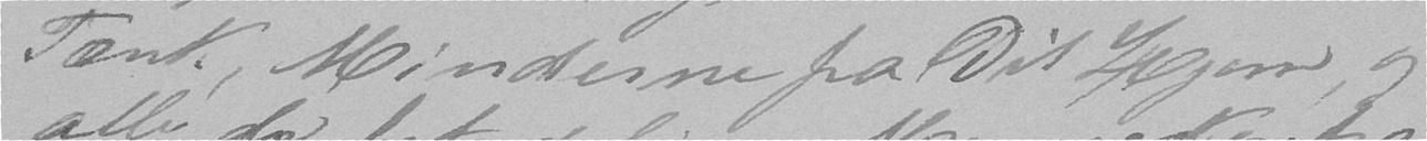Tænk, Minderne fra Dit Hjem og
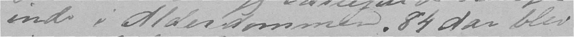inde i Alderdommen. 84 Aar blev
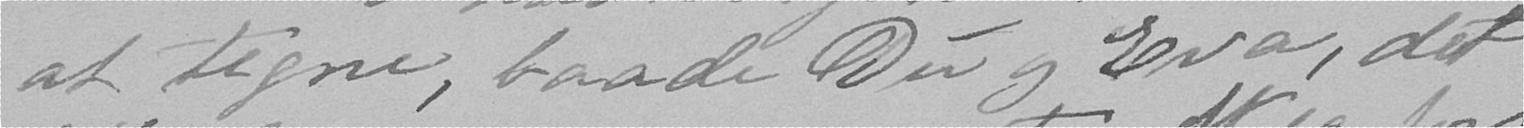at tegne, baade Du og Eva, det
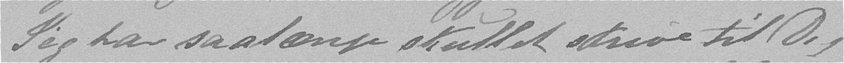Jeg har saalænge skullet skrive til Dig
